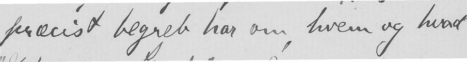præcist begreb har om, hvem og hvad
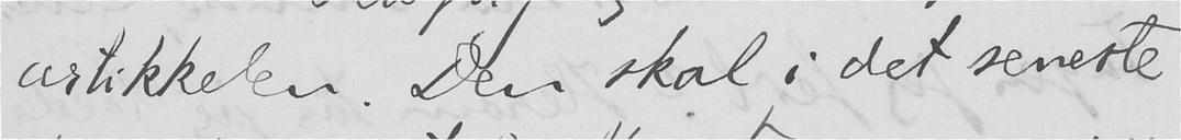artikkelen. Den skal i det seneste
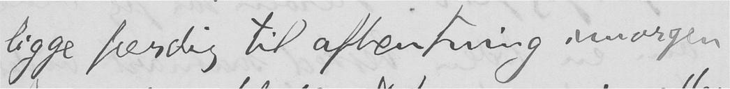ligge færdig til afhentning imorgen
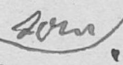som
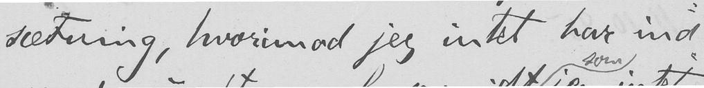sætning, hvorimod jeg intet har ind-
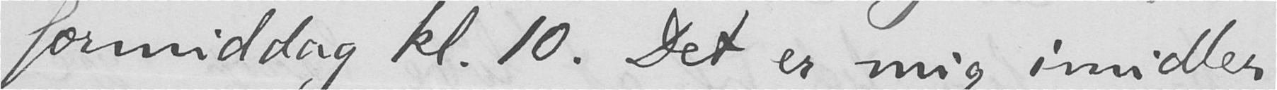formiddag kl. 10. Det er mig imidler-
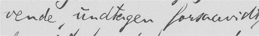vende, undtagen forsaavidt
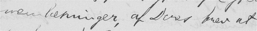nemlæsninger, af Deres brev at
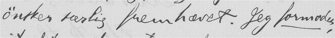ønsker særlig fremhævet. Jeg formoder,
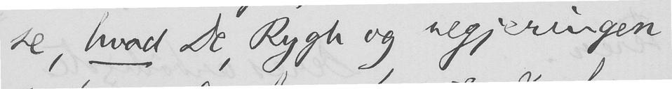se, hvad De, Rygh og regjeringen
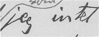jeg intet
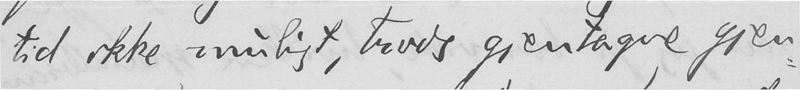tid ikke muligt, trods gjentagne gjen-
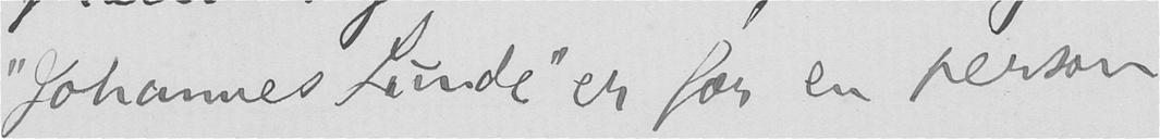"Johannes Lunde" er for en person.
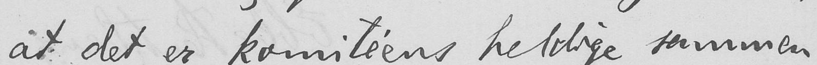at det er komitéens heldige sammen-
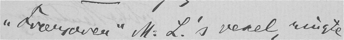"Tværsover", M. L.’s vexel, ringte
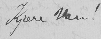Kjære Ven!
